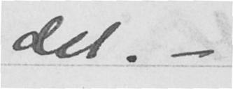det. -
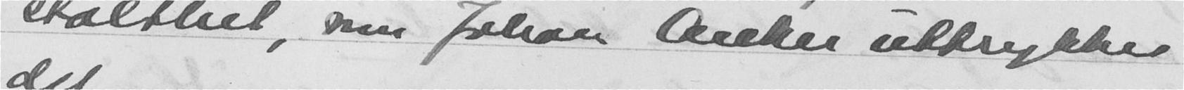stolthet, som Johan Anker uttrykker
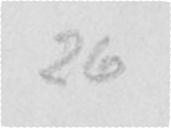26
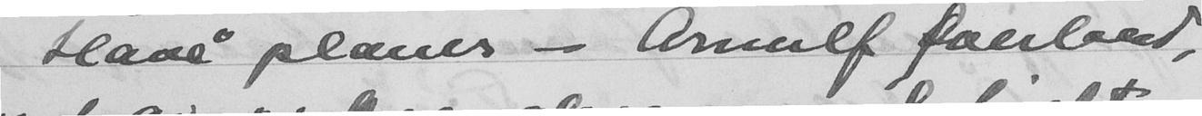og Have" planer - Arnulf Øverland,
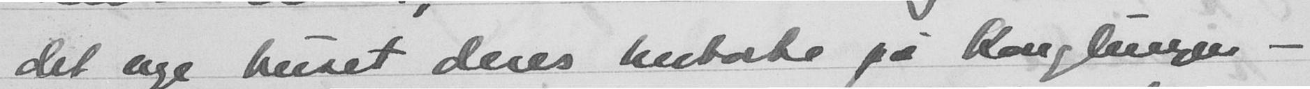det nye huset deres herborte på Konglungen -
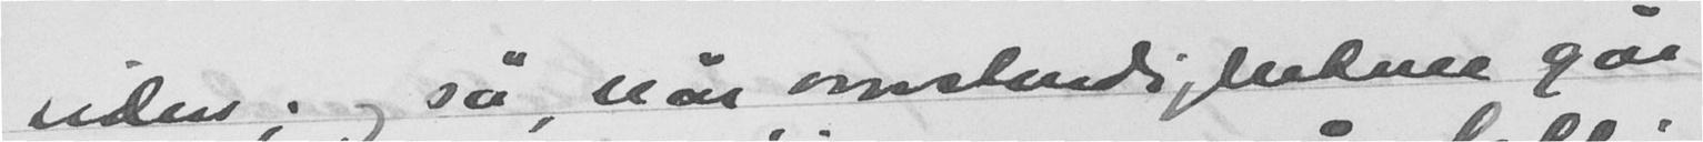siden; og så, når omstendighetene går
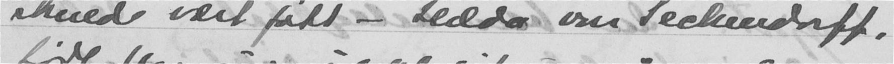skulde vært føtt - Lidda von Seckendorff,
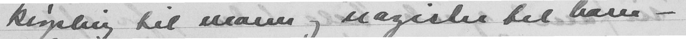krøpling til mann og nazister til barn -
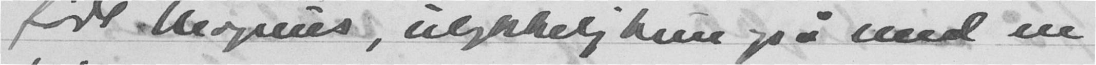født Magnus, ulykkelighet også med en
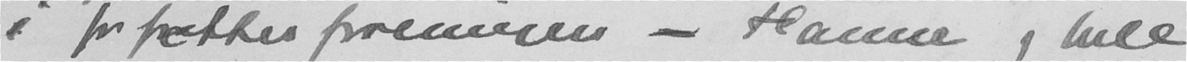i forfatterforeningen - Hanne og hele
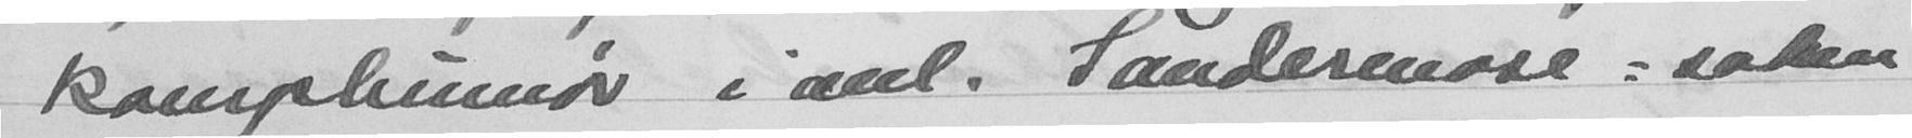kamphumør i anl. Sandemose-saken
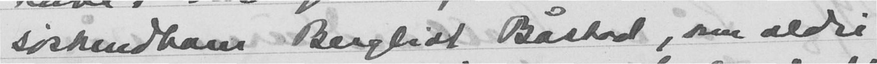søskenbarn Bergliot Båstad, som aldri
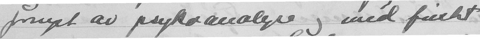fornyet av psykoanalyse og med fult
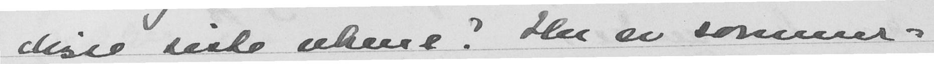disse siste ukene! Her er sommer-
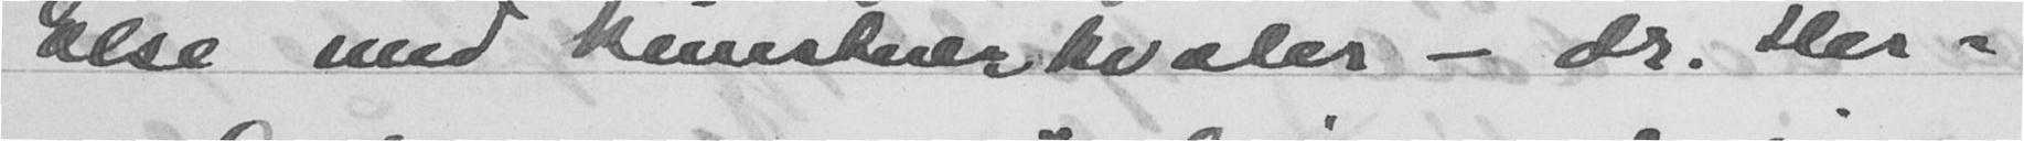Else med kunstnerkvaler - dr. Her-
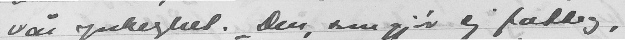vår ynkelighet. "Den, som gjør sig fattig,
